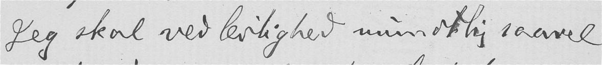Jeg skal ved leilighed mundtlig saavel
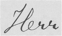Herr
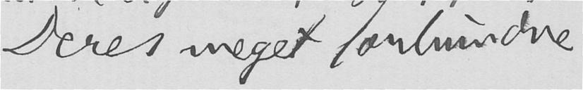Deres meget forbundne
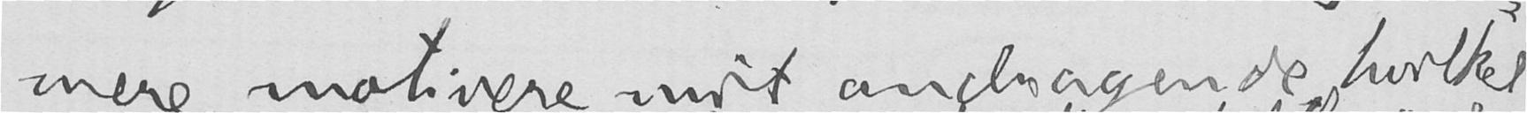mere motivere mit anliggende, hvilket
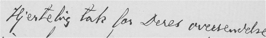Hjertelig tak for Deres oversendelse
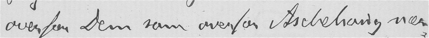overfor Dem som overfor Aschehoug nær-
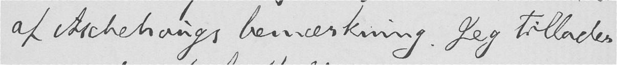af Aschehougs bemærkning. Jeg tillader
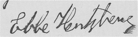Ebbe Hertzberg.
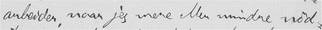arbeider, naar jeg mere eller mindre nød-
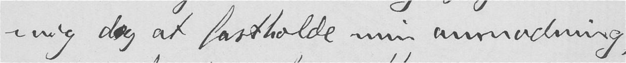mig dog at fastholde min anmodning,
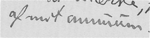af mit annuum.
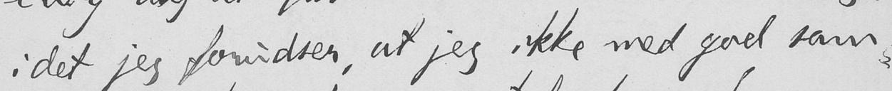idet jeg forudser, at jeg ikke med god sam-
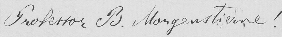Professor B. Morgenstierne!
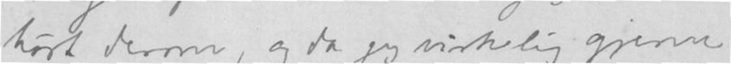hørt derom, og da jeg virkelig gjerne
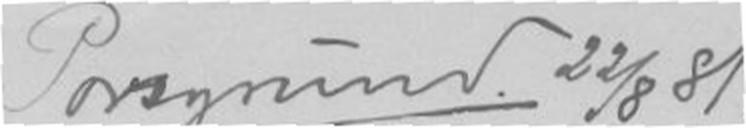Porsgrund 22/8 81.
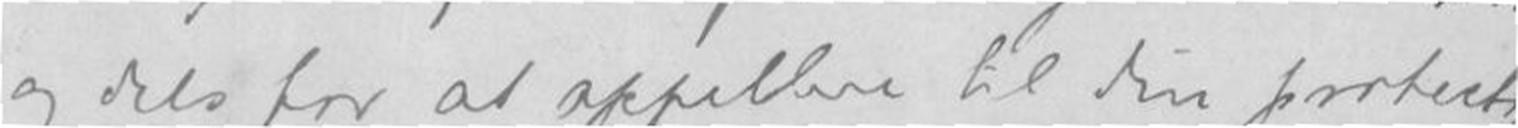og dels for at appellere til din protect
